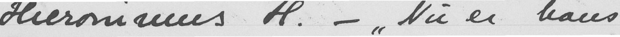Hieronimus H. — „Nu er hans
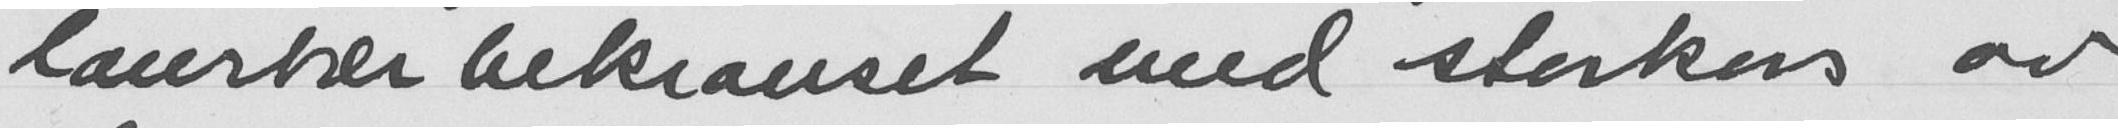laurbærbekranset med storkors av
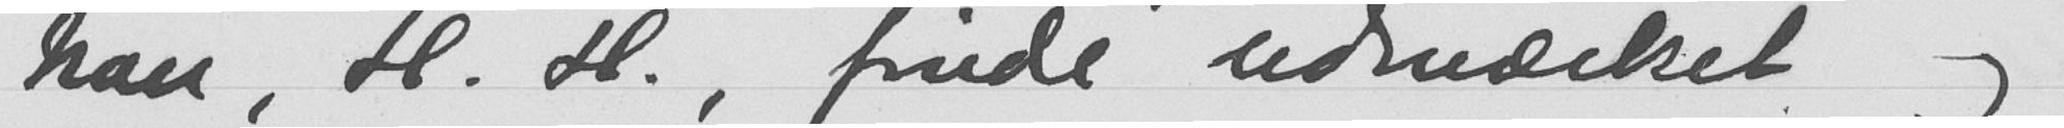han, H. H., finde udmerket og
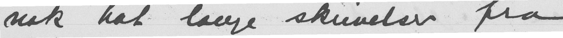nok hat lange skrivelser fra
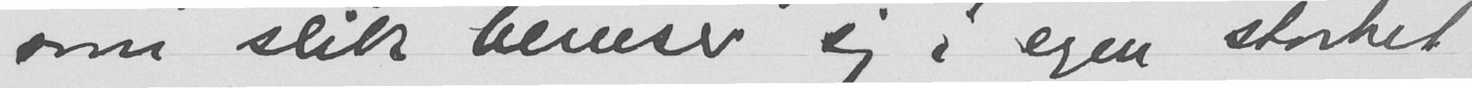som slik beruser sig i egen storhet.
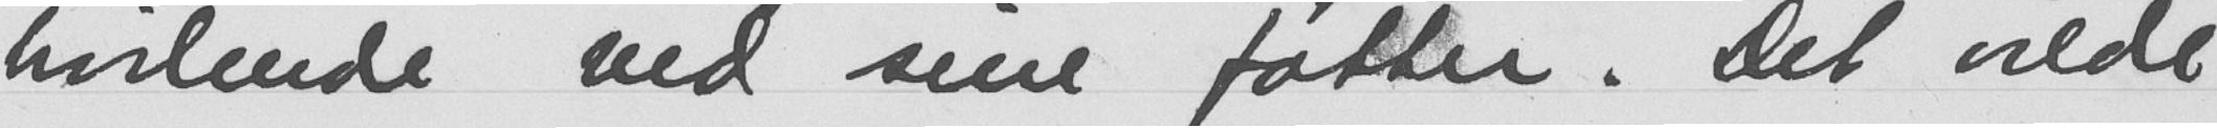hvilende ved sine føtter. Det vilde
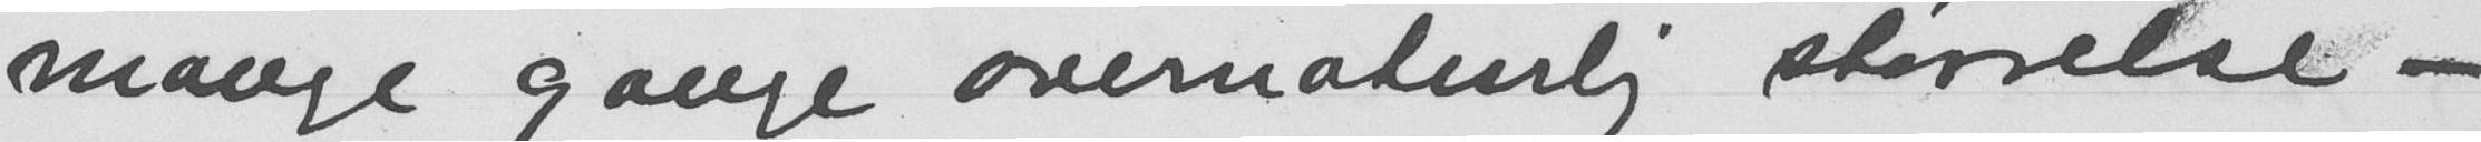mange gange overnaturlig størrelse -
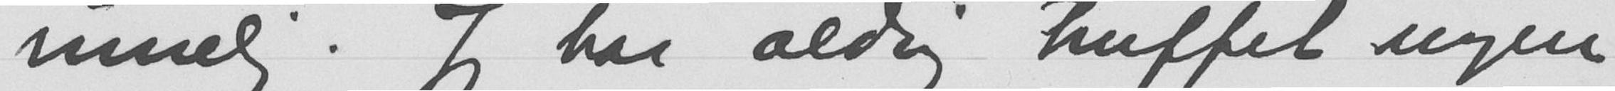rimelig. Jeg har aldrig truffet nogen
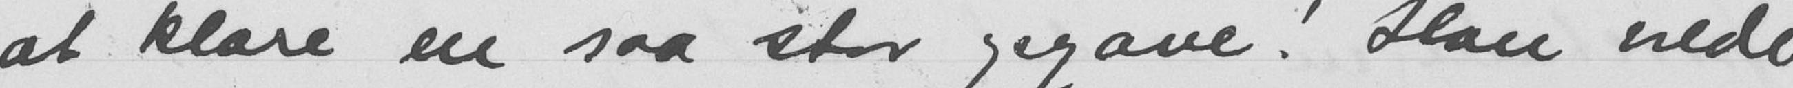at klare en saa stor opgave! Han vilde
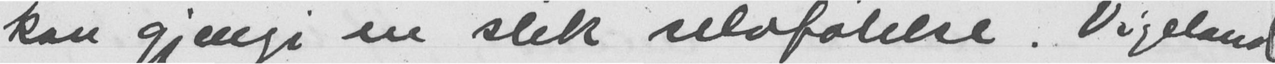kan gjengi en slik selvfølelse. Vigeland
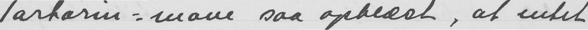Tartarin-mave saa opblæst, at intet
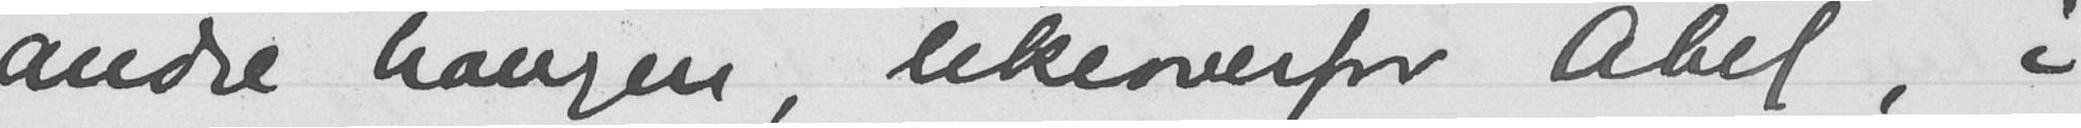andre haugen, likeoverfor Abel, i
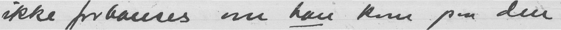ikke forbauses om han kom paa den
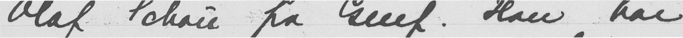Olaf Schou fra Genf. Han har
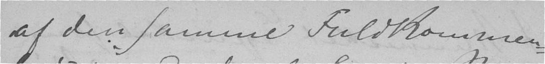af den samme Fuldkommen-
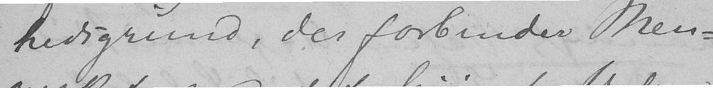hedsgrund, der forbinder Men-
